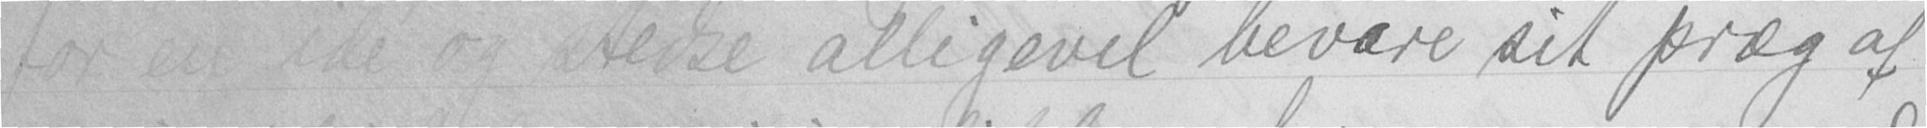for en idé og stedse alligevel bevare sit præg af
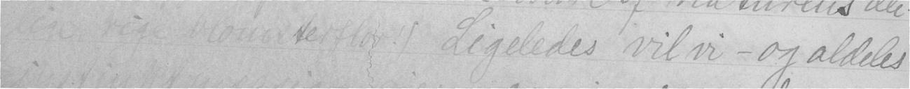lige rige blomsterflor!) Ligeledes vil vi - og aldeles
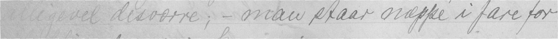alligevel desværre; - man staar næppe i fare for
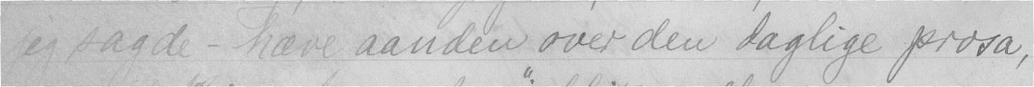jeg sagde - hæve aanden over den daglige prosa,
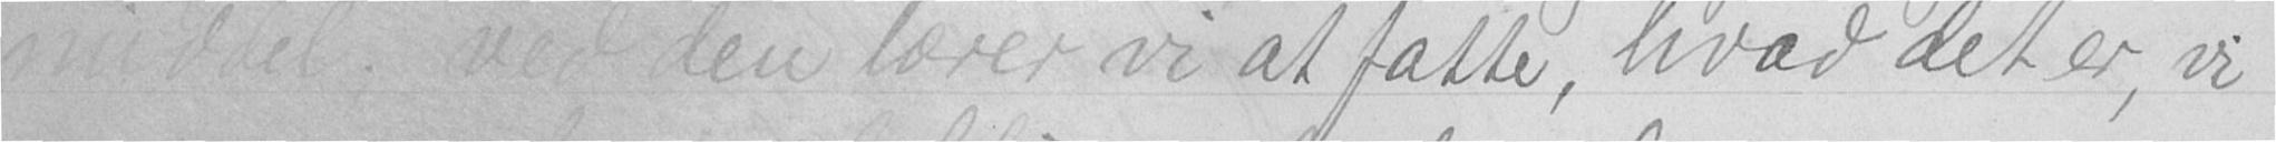middel, ved den lærer vi at fatte, hvad det er, vi
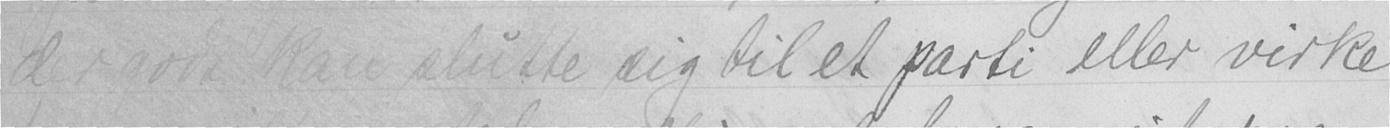der gode kan slutte sig til et parti eller virke
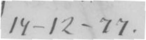14-12-77.
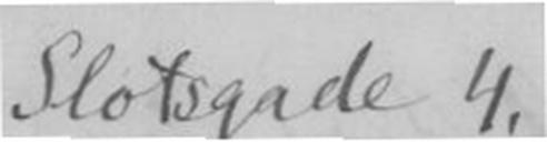Slotsgade 4,
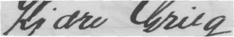Kjære Grieg
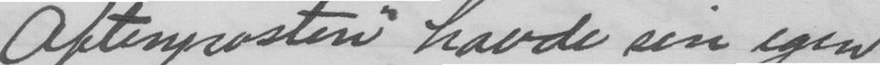"Aftenposten" havde sin egen
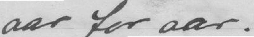aar for aar.
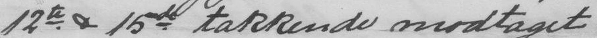12te & 15de takkende modtaget.
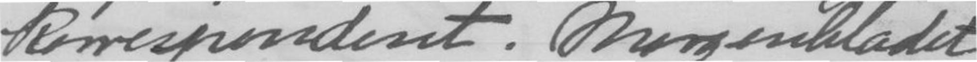korrespondent. Morgenbladet
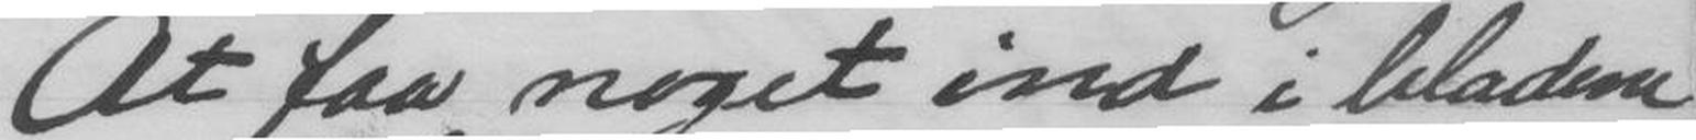At faa noget ind i bladern
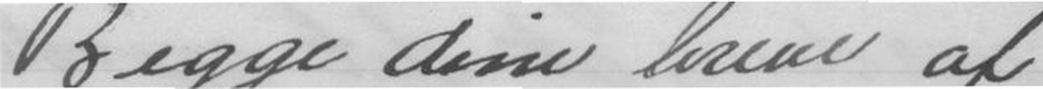Begge dine breve af
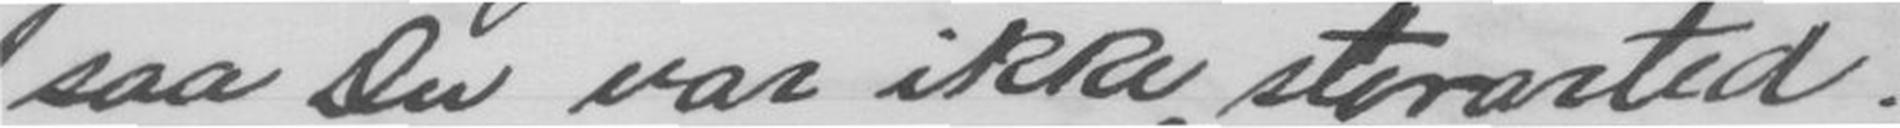saa Du var ikke storarted.
